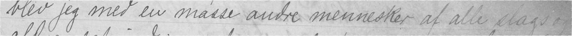blev jeg med en masse andre mennesker af alle slags og
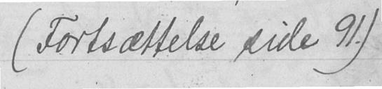(Fortsættelse side 91.)
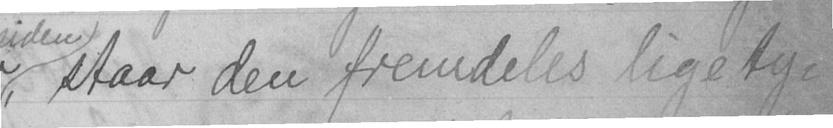, staar den fremdeles lige ty-
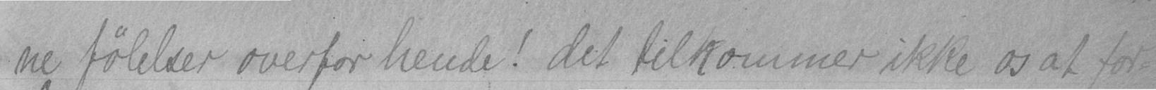ne følelser overfor hende! det tilkommer ikke os at for-
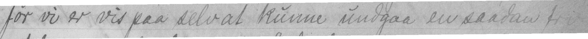før vi er vis paa selv at kunne undgaa en saadan fri-
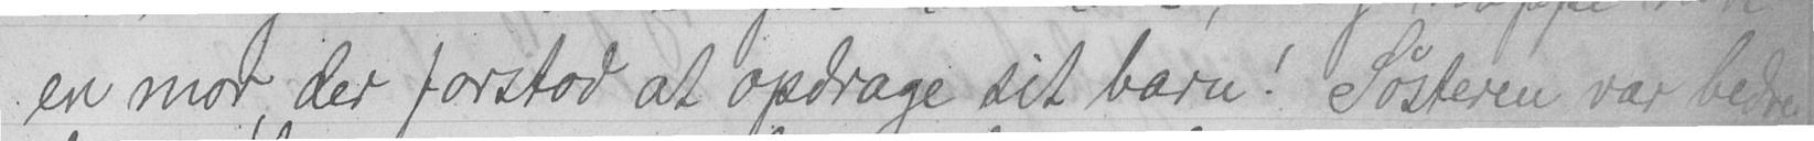en mor, der forstod at opdrage sit barn! Søsteren var bedre
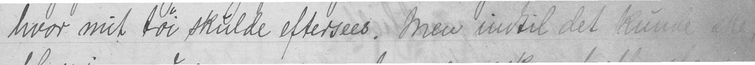hvor mit tøi skulde eftersees. Men indtil det kunde ske,
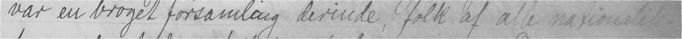var en broget forsamling derinde, folk af alle nationalite-
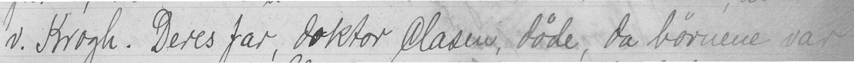v. Krogh. Deres far, doktor Clasen, døde, da børnene var
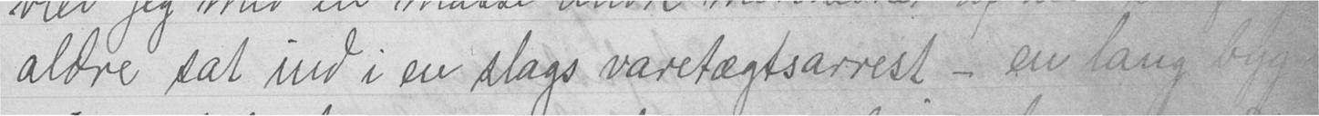aldre sat ind i en slags varetægtsarrest - en lang byg-
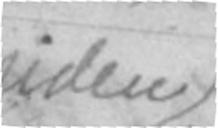siden
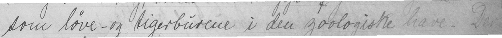som løve - og tigerburene i den zoologiske have. Der
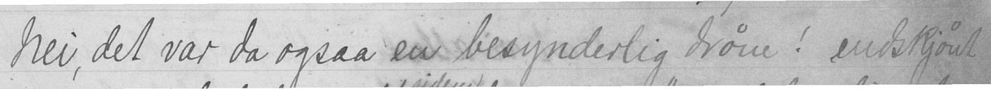Nei, det var da ogsaa en besynderlig drøm! endskjønt
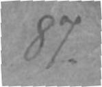87.
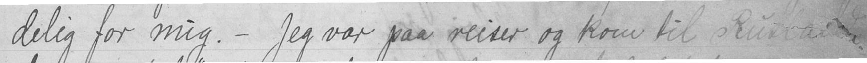delig for mig. - Jeg var paa reiser og kom til Rusland
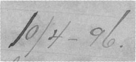10/4 - 96.
